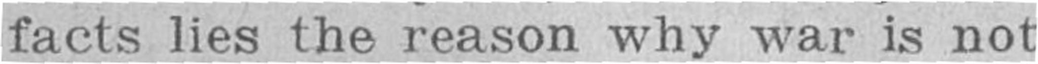facts lies the reason why war is not
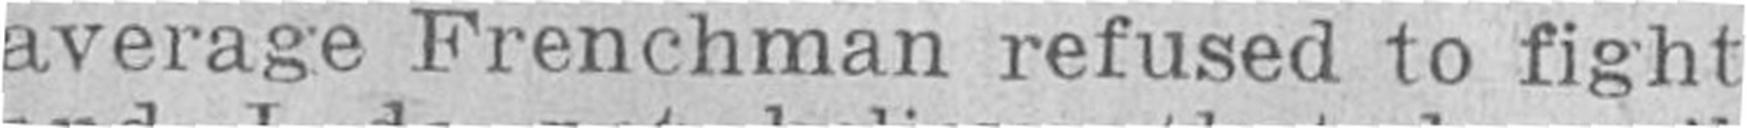average Frenchman refused to fight
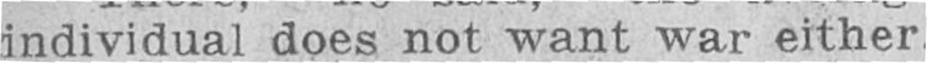individual does not want war either
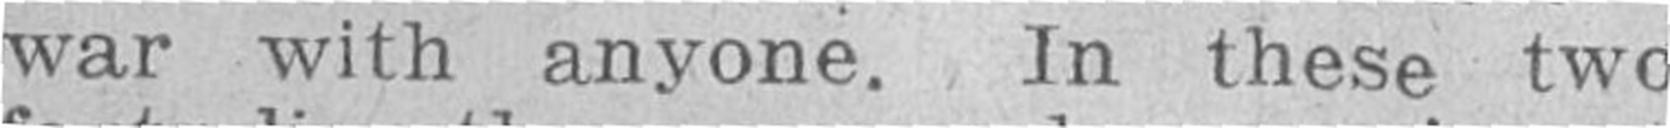war with anyone. In these two
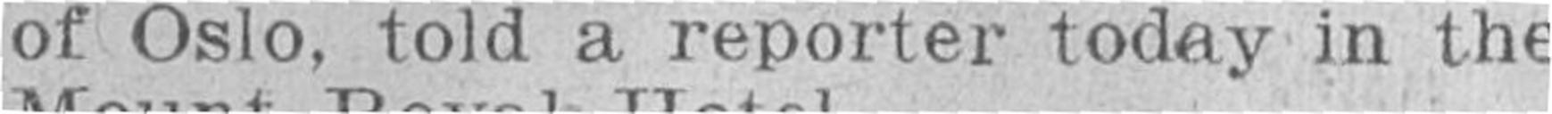of Oslo, told a reporter today in the
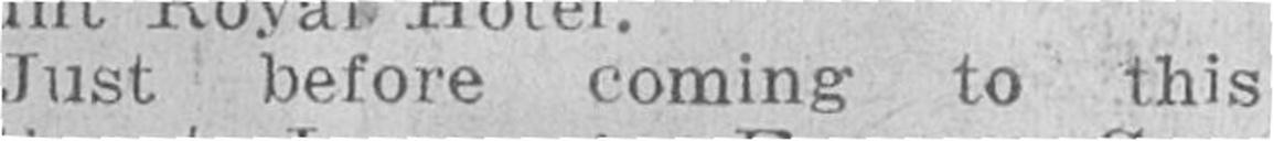"Just before coming to this
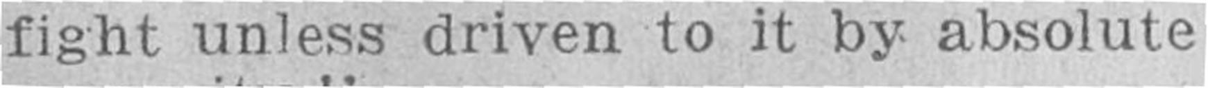fight unless driven to it by absolute
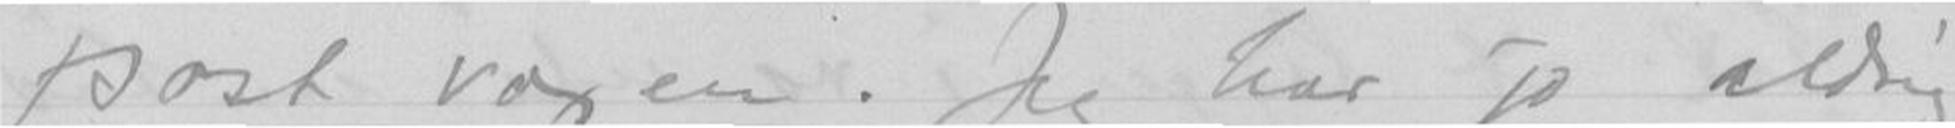post voxen. Jeg har jo aldrig
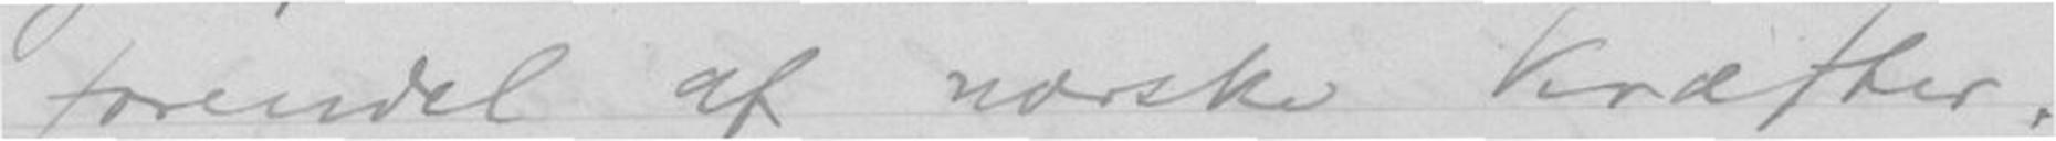forendel af norske kræfter.
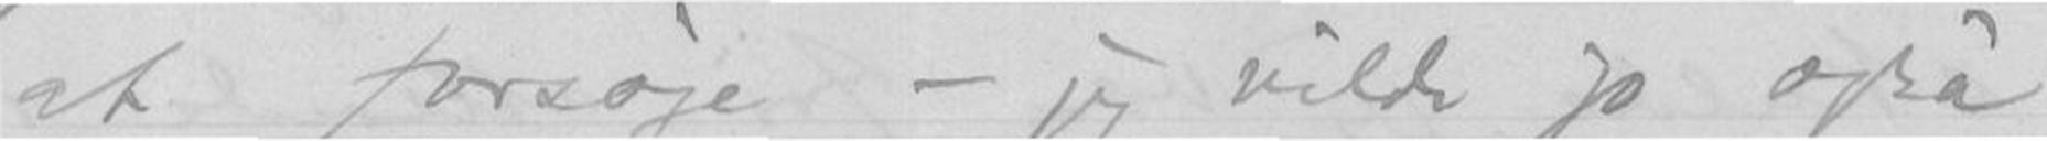at forsøge - jeg vilde jo også
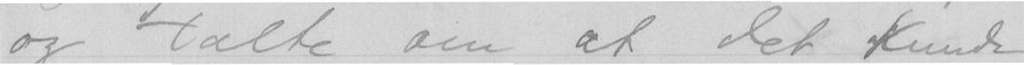og talte om at det kunde
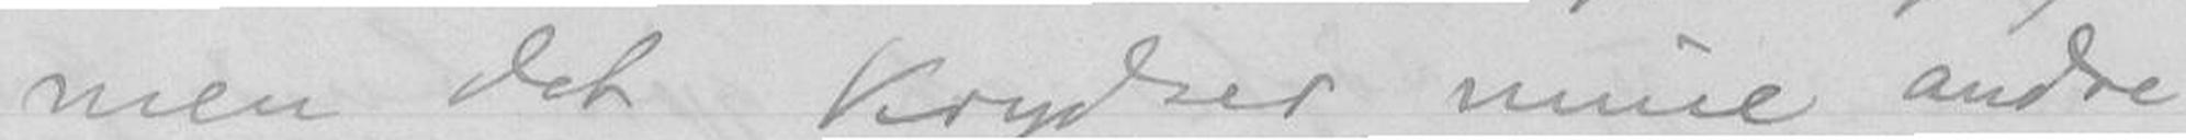men det krydser mine andre
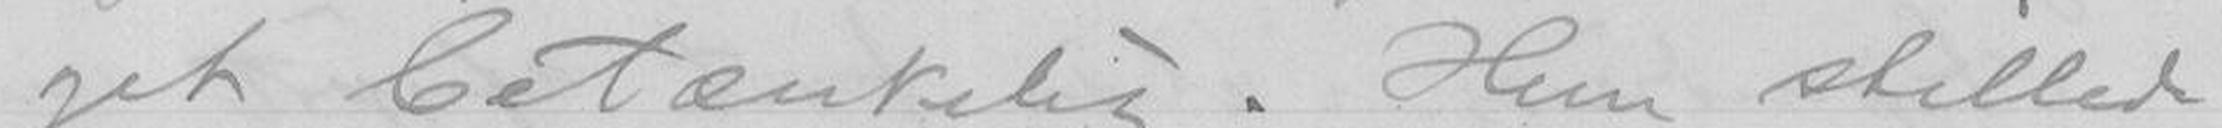get betænkelig. Hun stillede
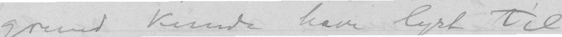grund kunde have lyst til
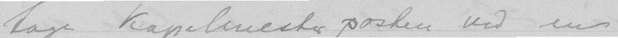tage kapelmester posten ved en
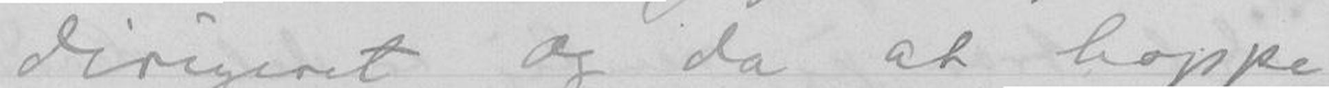dirigeret og da at hoppe
